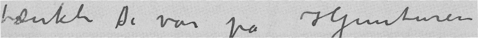tænkte De var på Hjemturen
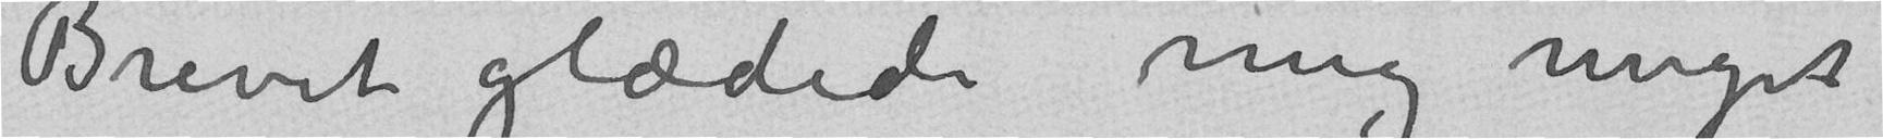Brevet glædede mig meget
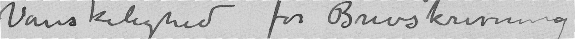Vanskelighed for Brevskriving
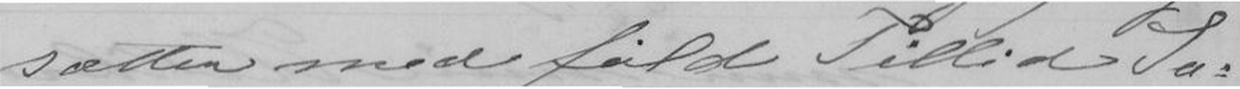sætter med fuld Tillid Sa-
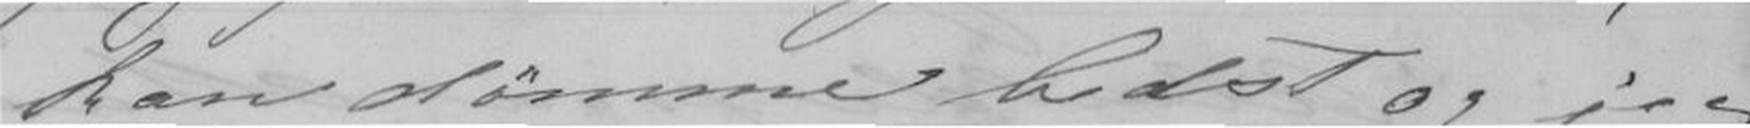kan dømme bedst og jeg
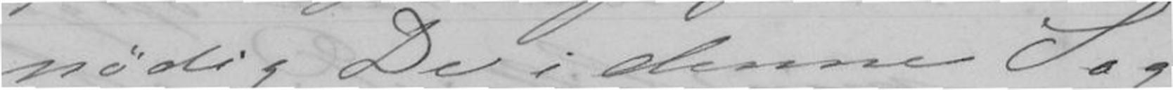nødig De i denne Sag
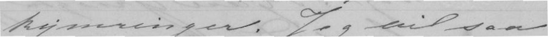kymringer. Jeg vil saa
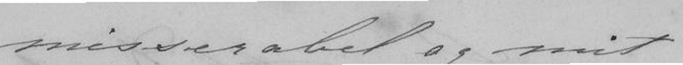misserabel og mit
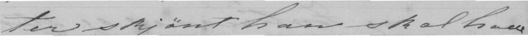ter skjønt han skal have
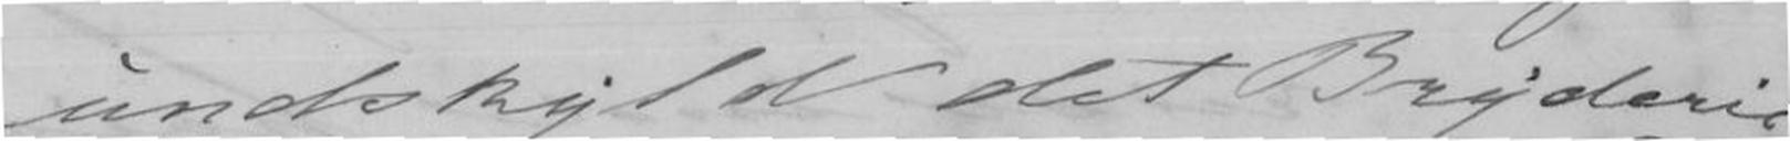undskyld det Bryderi
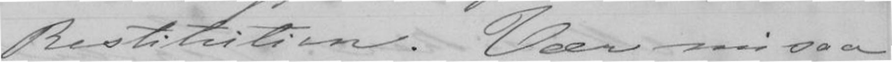Restitution. Vær nu saa
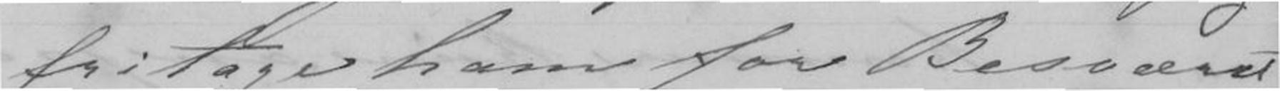fritage ham for Besværet
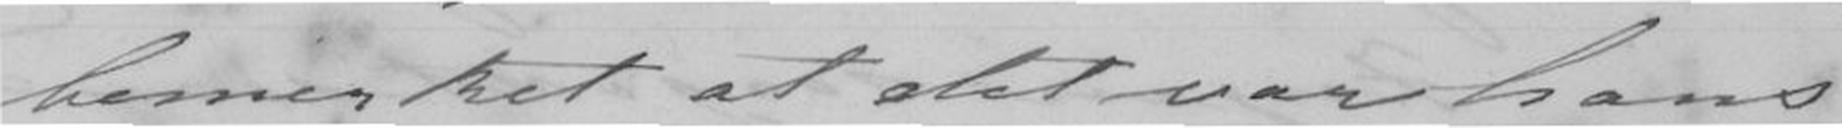bemerket at det var hans
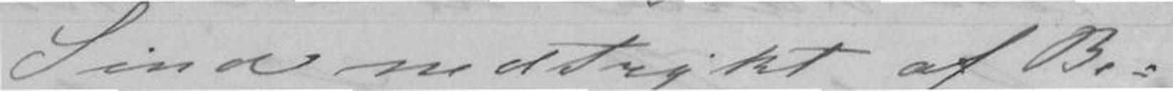Sind nedtrykt af Be-
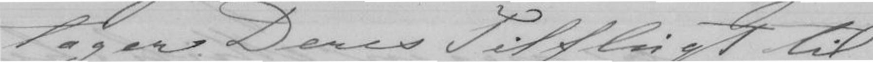tager Deres Tilflugt
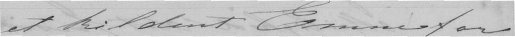et kildent Emne for
